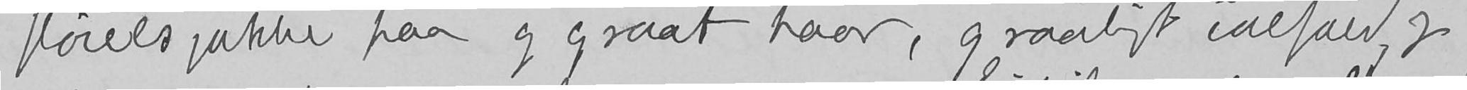fløielsjakke paa og graat haar, graaligt ialfald, og
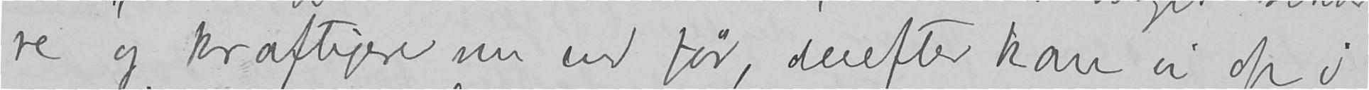re og kraftigere nu end før, derefter kom vi op i
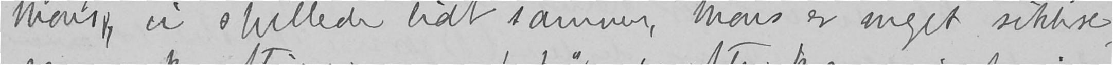Mons, vi spillede lidt sammen, Mons er meget sikkre-
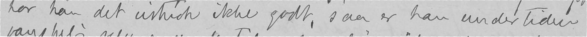har han det vistnok ikke godt, saa er han undertiden
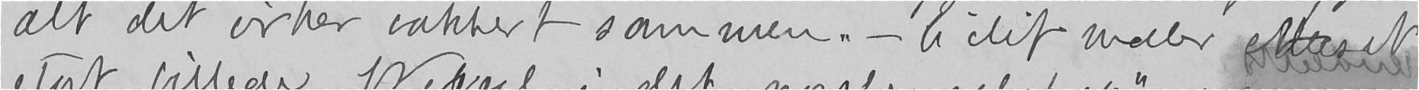alt det virker vakkert sammen. - Eilif maler ellers et
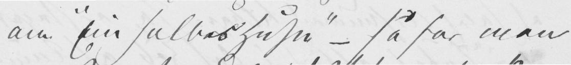om "Ein halbes Huhn" - så far man
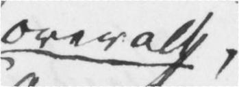overalt,
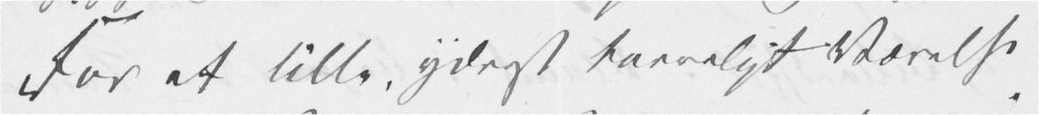For et lille, yderst tarveligt Værelse
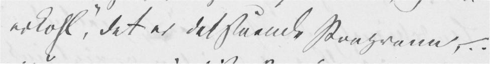erkofl," det er det stående Program,
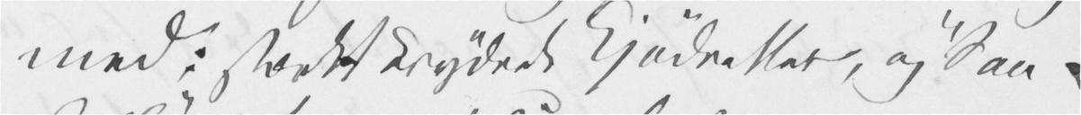med: stærkt krydrede Kjødretter, og "Sau-
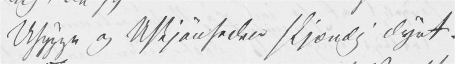Uhygge og Uskjønheder skjændig dyrt.
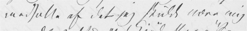modsatte af det jeg skulde nære mig
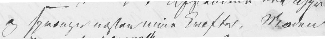og sprænger næsten mine Kræfter, Maden
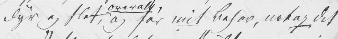dyr og slet, og for mit Behov, netop det
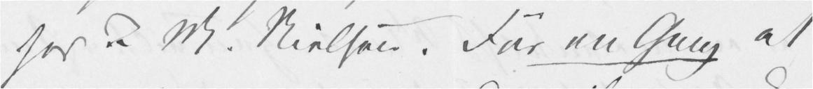hos I. M. Nielsen. For en Gang at
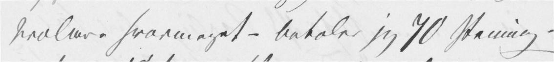trollere hvormeget – betaler jeg 70 Penning -
